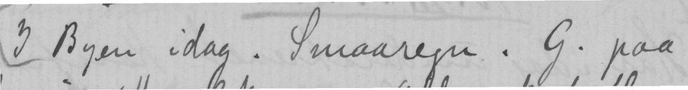I Byen idag. Smaaregn. G. paa
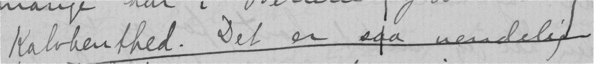Kalvbenthed. Det er saa uendelig
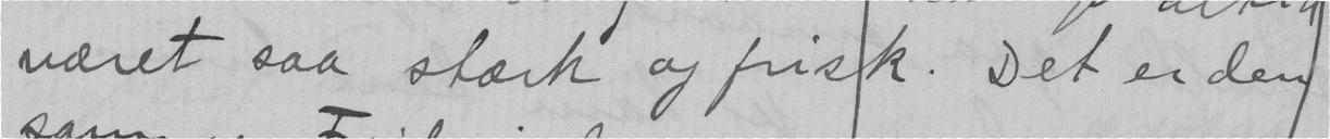været saa stærk og frisk. Det er den
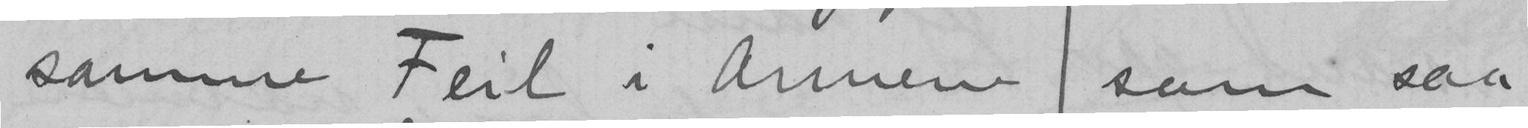samme Feil i armen som saa
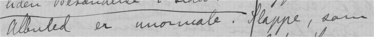albuled er unormale. Slappe, som
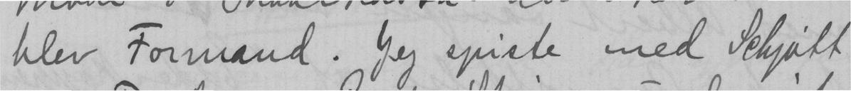blev Formand. Jeg spiste med Schjøtt,
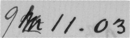9  11.03
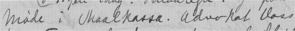møde i Maalkassa. Advokat Voss
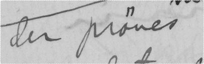der prøves
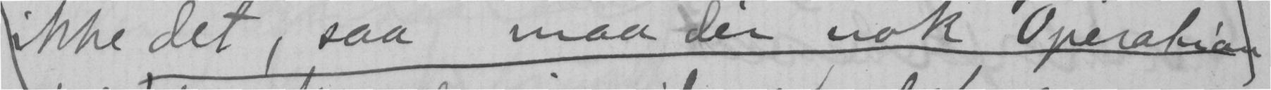ikke det, saa maa der nok Operation
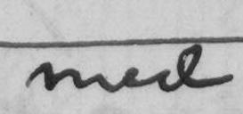med
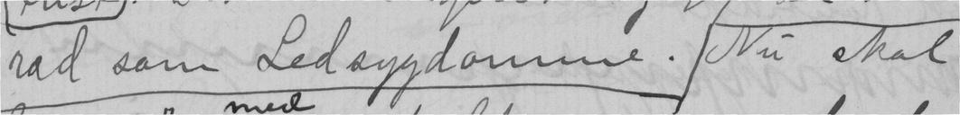ræd som Ledsygdomme. Nu skal
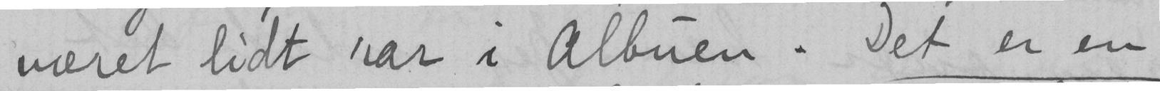været lidt rar i Albuen. Det er en
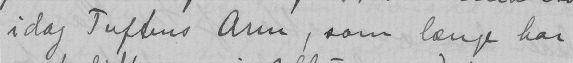idag Tuftens arm, som længe har
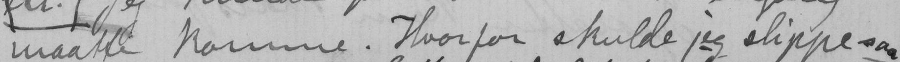maatte komme. Hvorfor skulde jeg slippe saa
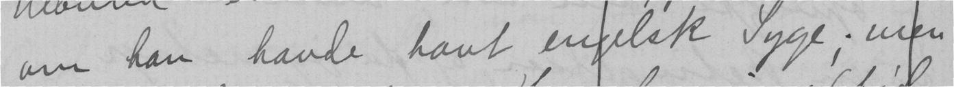om han havde havt engelsk syge; men
